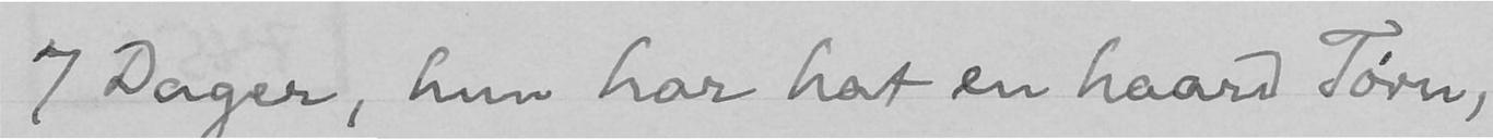7 Dager, hun har hat en haard Tørn,
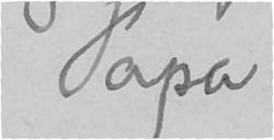Papa
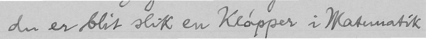du er blit slik en Kløpper i Matematik
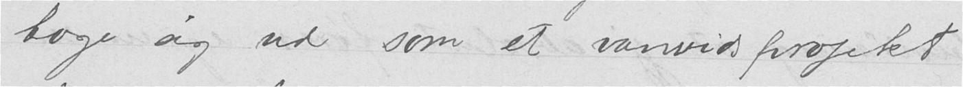tage sig ud som et vanvidsprojekt
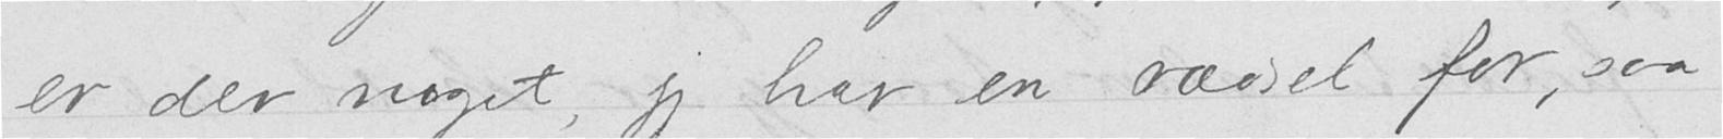er der noget, jeg har en rædsel for, saa
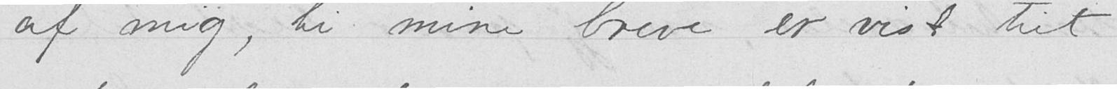af mig, ti mine breve er vist tit
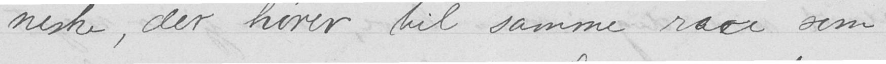neske, der hører til samme race som
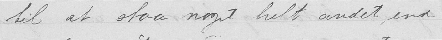til at staa noget helt andet, end
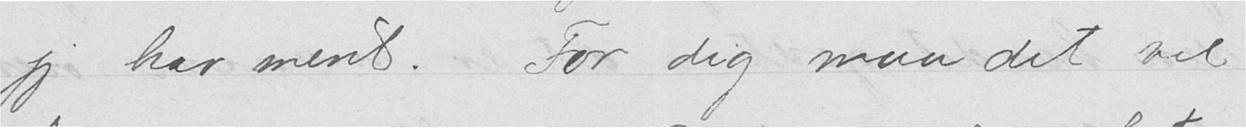jeg har ment. For dig maa det vel
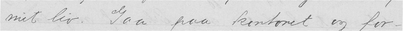mit liv. Gaa paa kontoret og for-
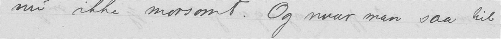nu ikke morsomt. Og naar man
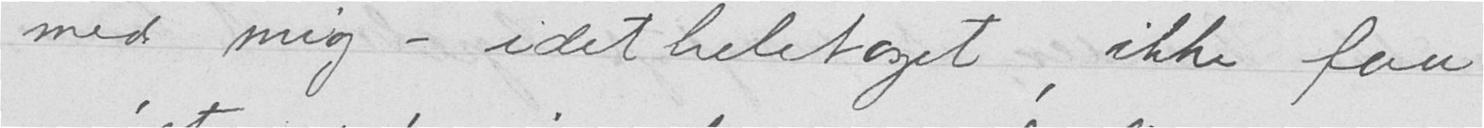med mig - idetheletaget, ikke faa
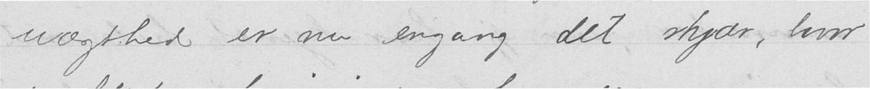uægthed er nu engang det skjær, hvor
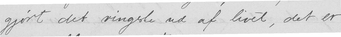gjort det ringeste ud af livet, det er
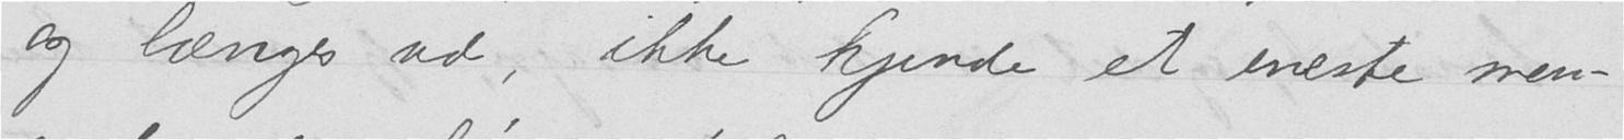og længes ud, ikke kjende et eneste men-
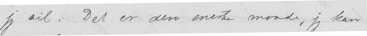jeg vil. Det er den eneste maade, jeg kan
